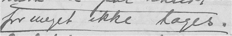for meget ikke tages.
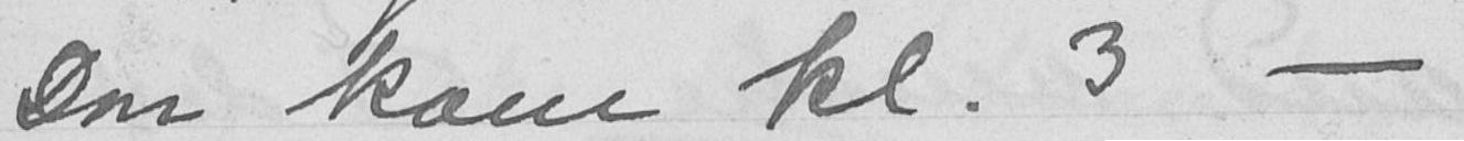Don kom kl. 3 -
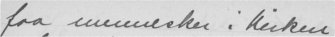faa mennesker i kirken
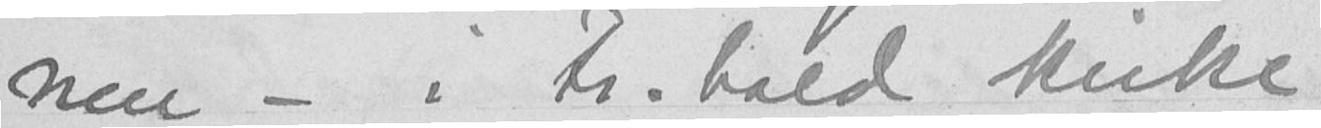men - i Fr.hald kirke,
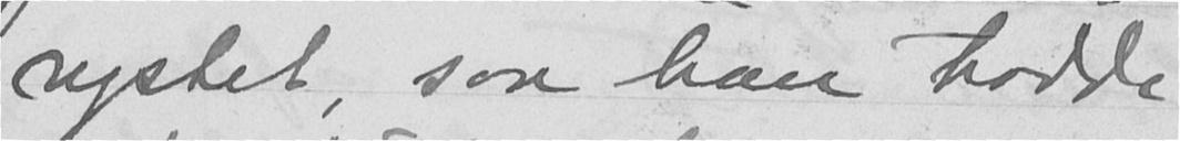rystet, saa han trodde
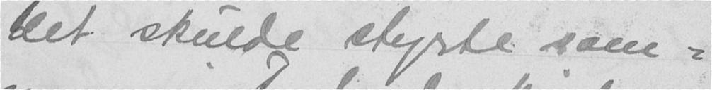det skulde styrte sam-
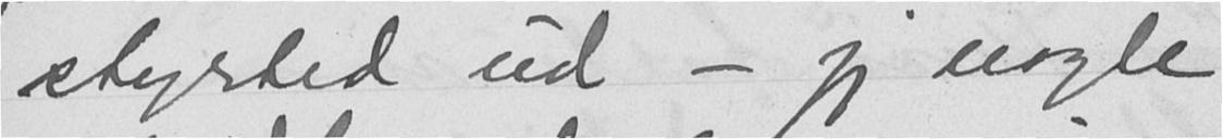styrted ud - jeg nogle
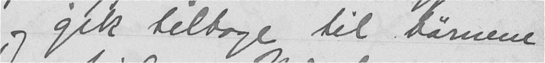og gik tilbage til børnene
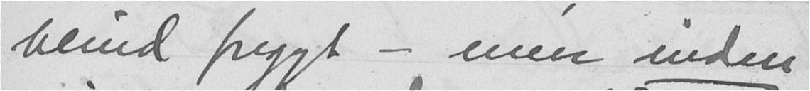blind frygt - men inden
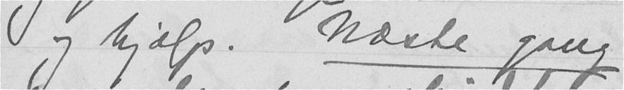og hjalp. Næste gang
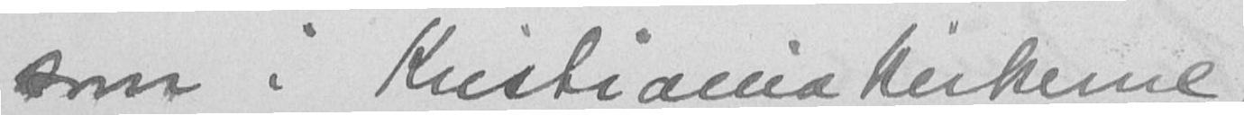som i Kristianiakirkerne,
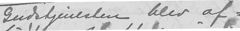Gudstjenesten blev af-
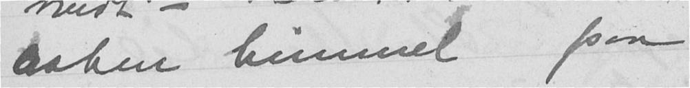aaben himmel paa
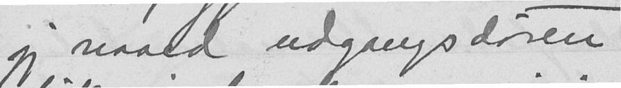jeg naaed udgangsdøren
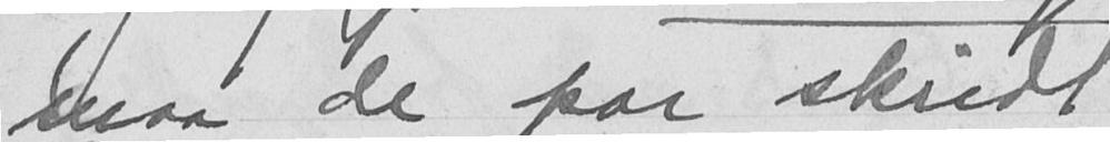maa de par skridt
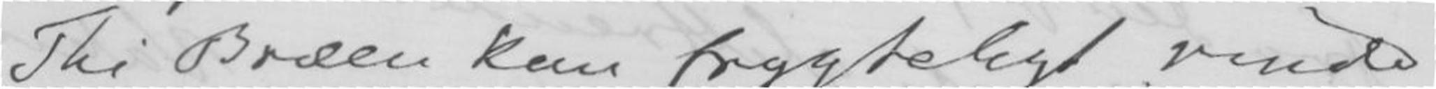Thi Bræen kan frygteligt vinde
